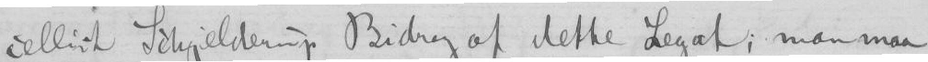cellist Schjelderup Bidrag af dette Leget; man maa
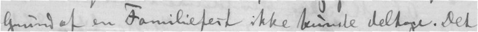Grund af en Familiefest ikke kunde deltage. Det
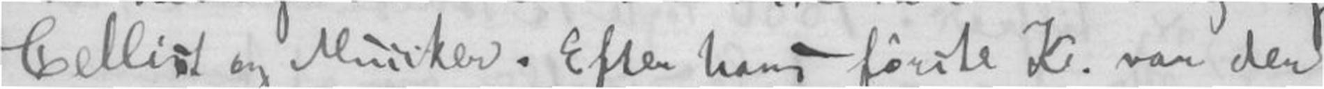Cellist og Musiker. Efter hans første K. var der
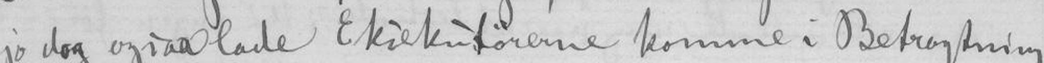jo dog ogsaa lade Eksekutørerne komme i Betragtning.
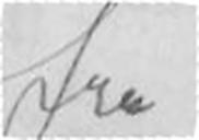fra
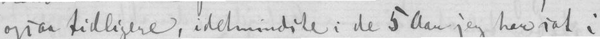ogsaa tidligere, idetmindste i de 5 Aar jeg her sat i
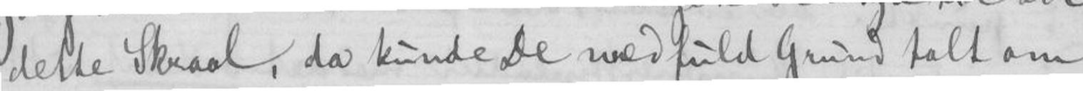dette Skraal, da kunde De med fuld Grund talt om
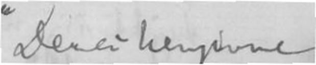Deres hengivne
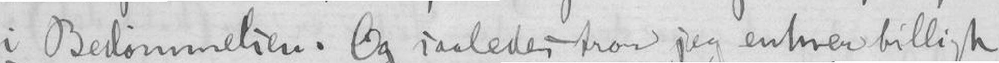i Bedømmelsen. Og saaledes tror jeg enhver billigt
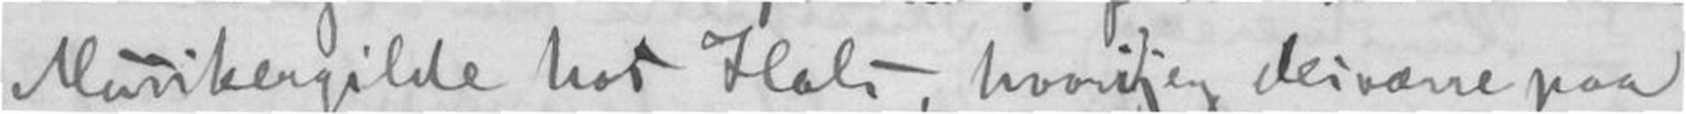Musikergilde hos Hals, hvori jeg desværre paa
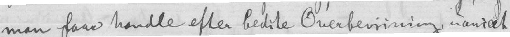man faar handle efter bedste Overbevisning uansæt
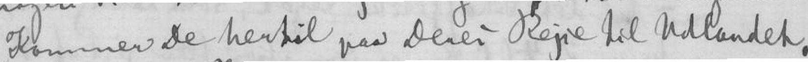Kommer De hertil paa Deres Rejse til Udlandet,
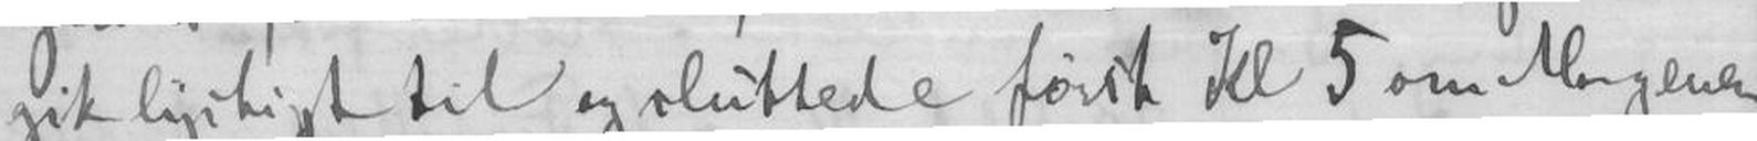gik lystigt til og sluttede først Kl 5 om Morgenen,
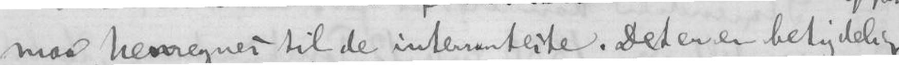maa herregnes til de interranteste?. Det er en betydelig
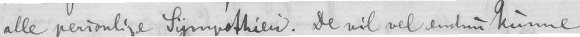alle personlige Sympathier. De vil vel endnu kunne
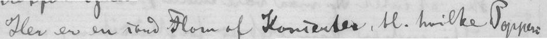Her er en sand Flom af Koncerter, bl. hvilke Popperi
